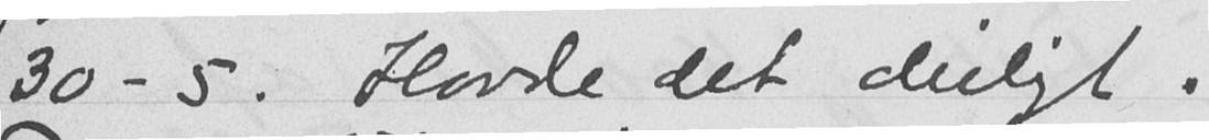30-5. Havde det deiligt.
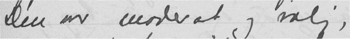Den var moderat og rolig,
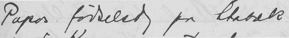Papas fødselsdag paa Stabæk
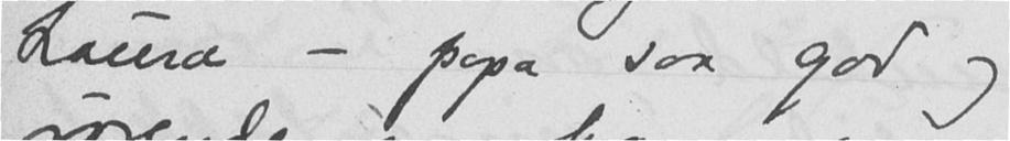Laura - papa saa god og
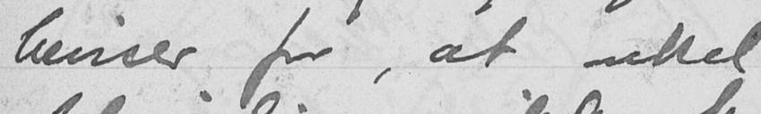beviser for, at onkel
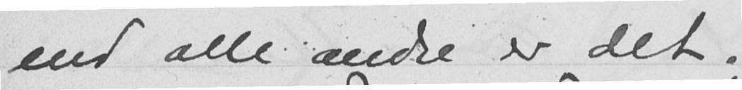end alle andre er det.
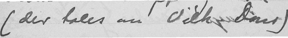(der tales om Vilh Dons)
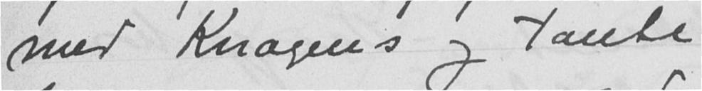med Knagens og tante
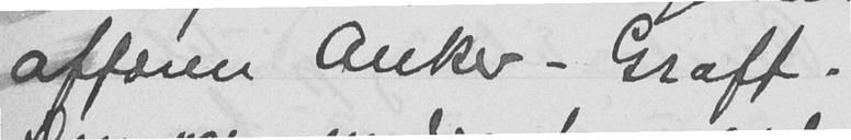affæren Anker-Graff.
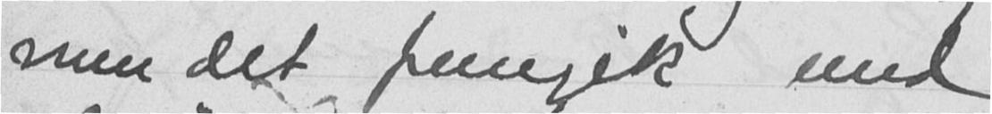men det fremgik med
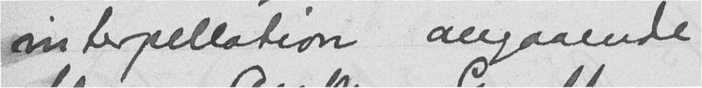interpellation angaaende
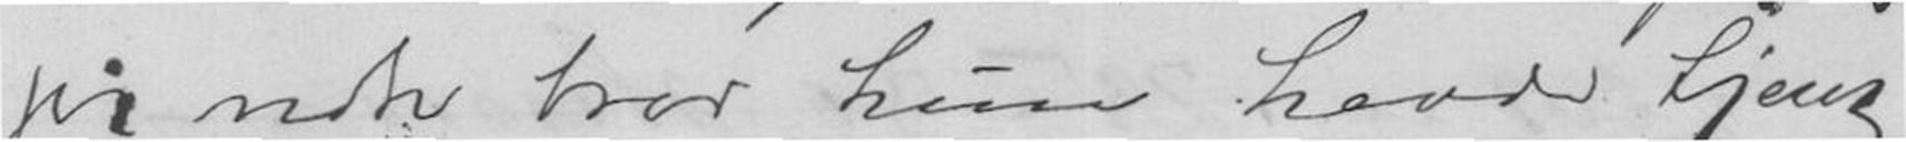jeg nok tror hun havde tjent
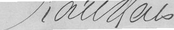Karl Hals
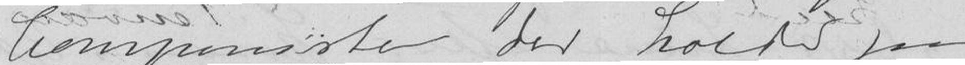Componister der holde paa
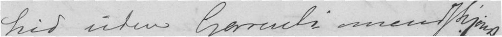hid uden Garanti omendskjøndt
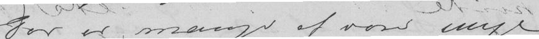Der er mange af vore unge
